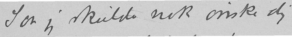Saa jeg skulde nok ønske dig
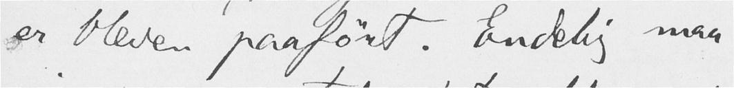er bleven paaført. Endelig maa
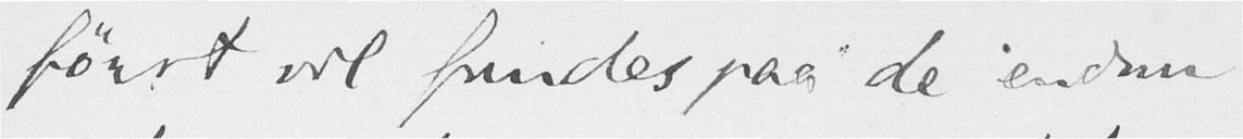først vil findes paa de endnu
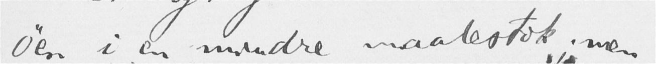øen i en mindre maalestok; men
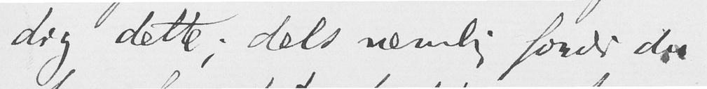dig dette; dels nemlig fordi du
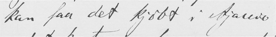kan faa det kjøbt i Ajaccio.
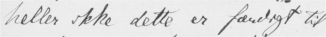heller ikke dette er færdigt til
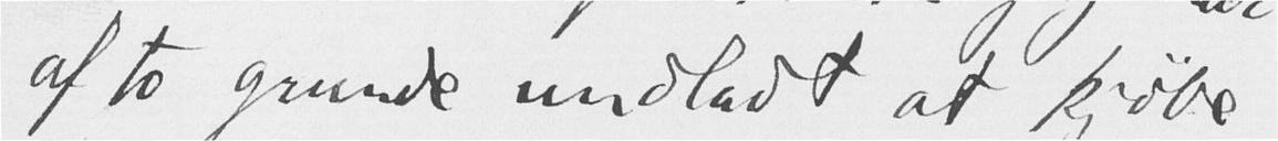af to grunde undladt at kjøbe
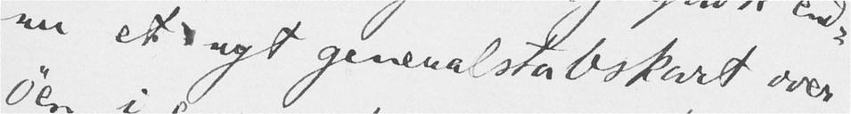nu et nyt generalstabskart over
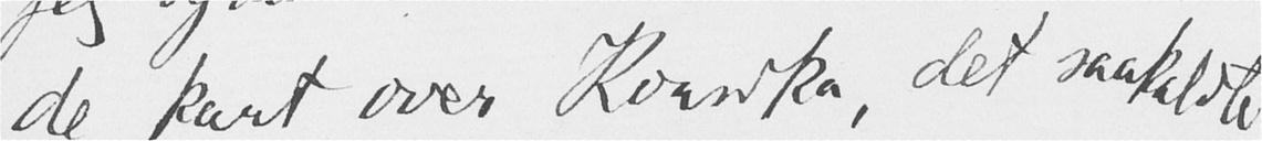de kart over Korsika, det saakaldte
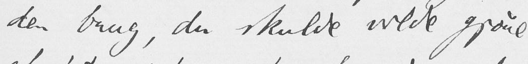den brug, du skulde vilde gjøre
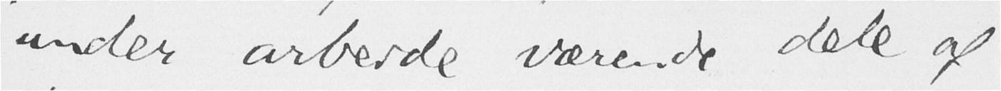under arbeide værende dele af
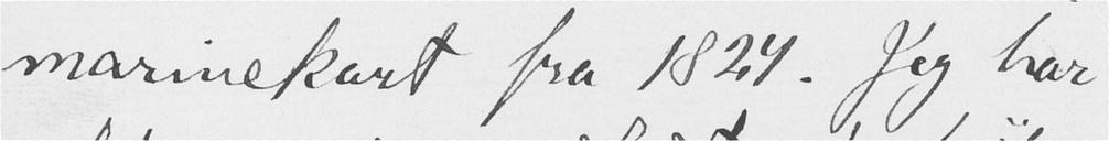marinekart fra 1824. Jeg har
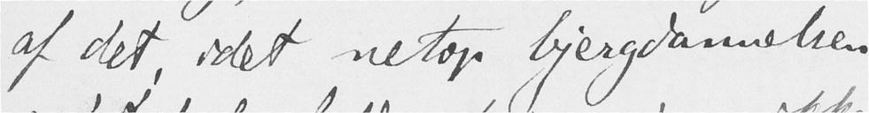af det, idet netop bjergdannelsen
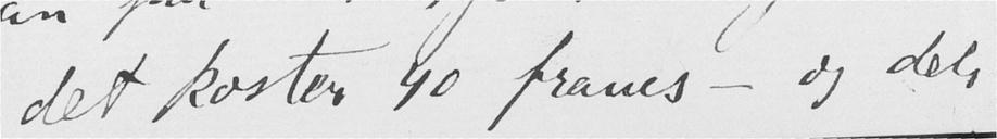– det koster 40 francs – og dels
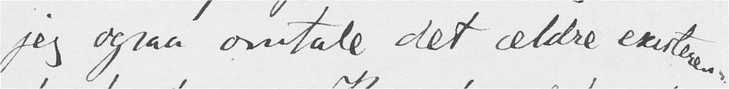jeg ogsaa omtale det ældre existeren-
